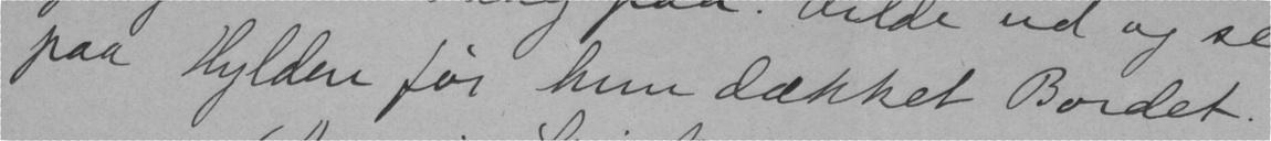paa Hylden før hun dækket Bordet.
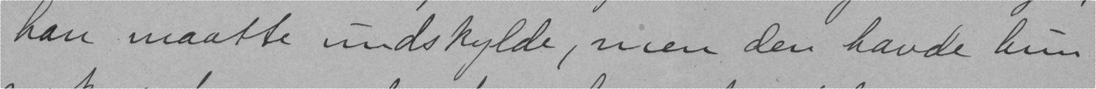han maatte undskylde, men den havde hun
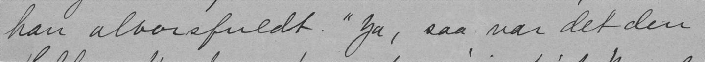han alvorsfuldt. "Ja, saa var det den
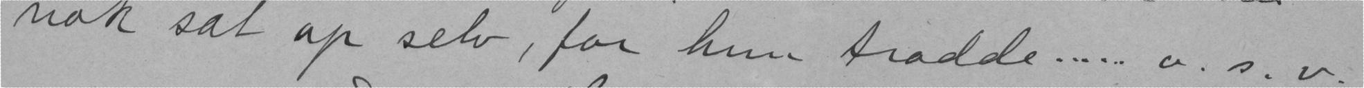nok sat op selv, for hun trodde ... o. s.v.
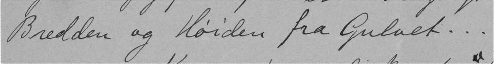Bredden og Høiden fra Gulvet..."
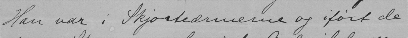Han var i Skjorteærmerne og iført de
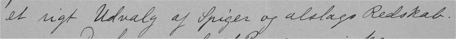et rigt Udvalg af Spiger og alslags Redskab.
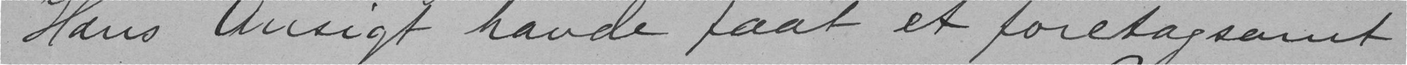Hans Ansigt havde faat et foretagsamt
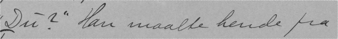"Du? Han maalte hende fra
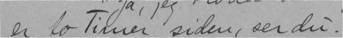er to Timer siden, ser du."
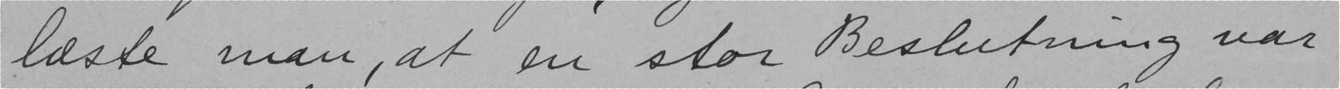læste man, at en stor Beslutning var
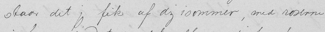staar det jeg fik af dig i sommer, med roserne
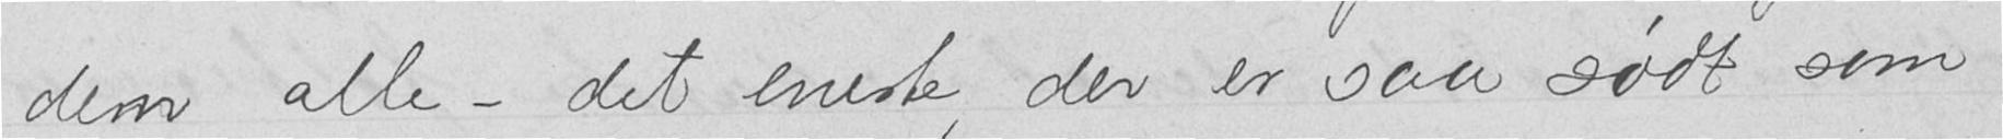dem alle - det eneste, der er saa sødt som
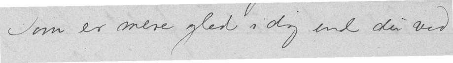som er mere glad i dig end du ved.
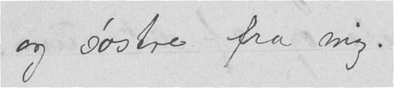og søstre fra mig.
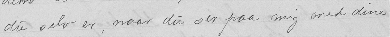du selv er, naar du ser paa mig med dine
